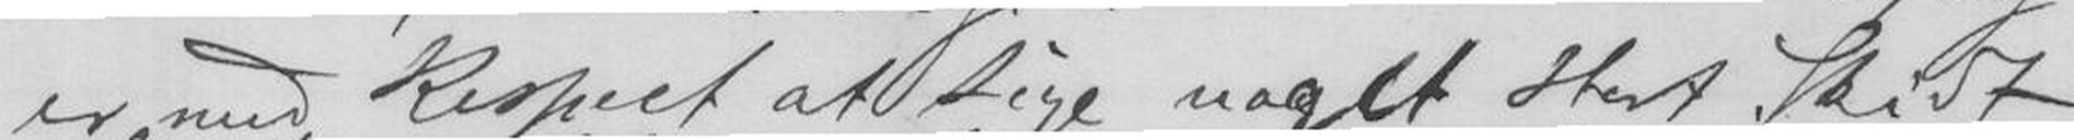er med Respect at sige noget stort Skidt
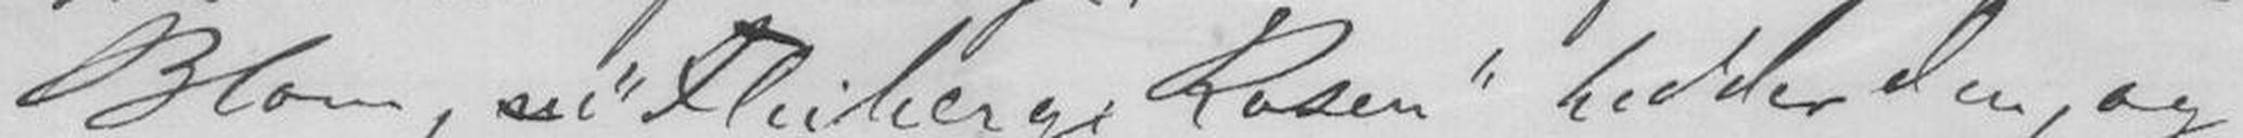Blom, "Fleiberg Rosen" hedder den, og
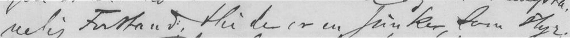velig Forstand; thi der er en Junker, som Styr-
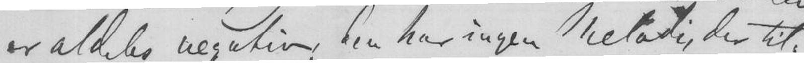er aldeles negativ; den har ingen Melodi, der til
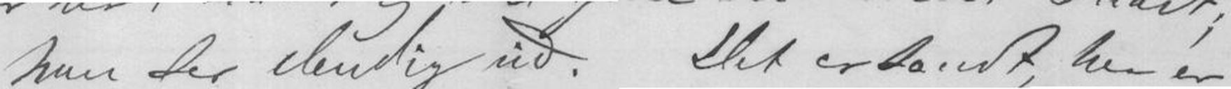han ser elendig ud. Det er sandt, her er
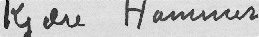Kjære Hammer
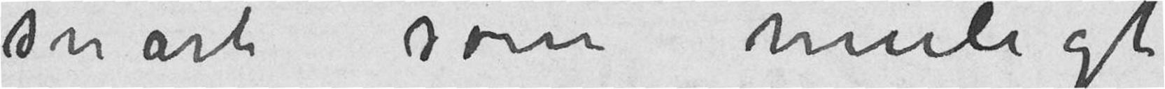snart som muligt
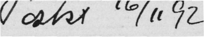16/11 92
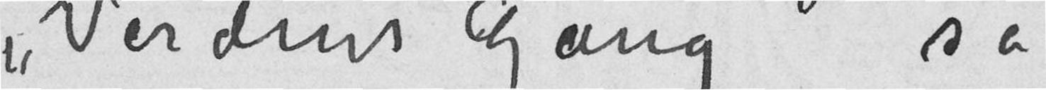i «Verdens Gang» så
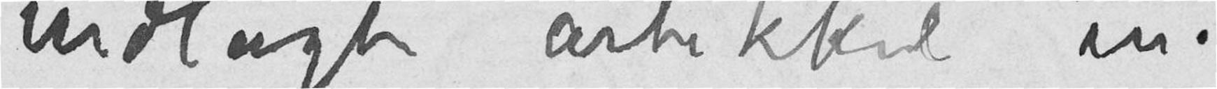vedlagte artikkel in
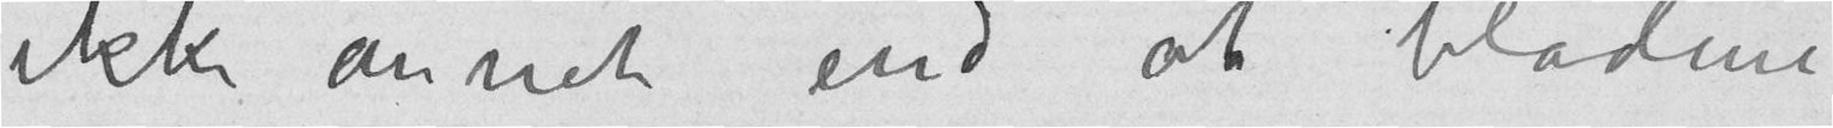ikke annet end at bladene
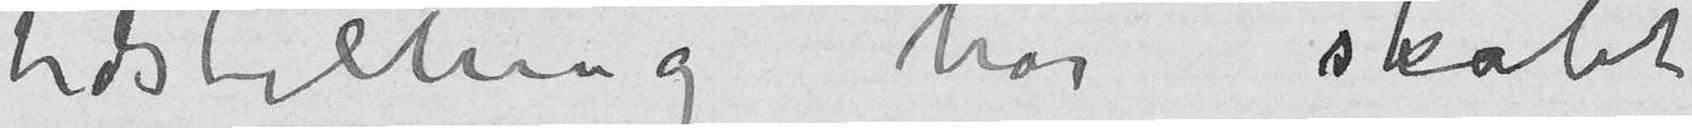udstilling har skabt
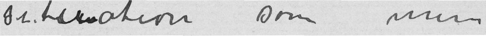situation som min
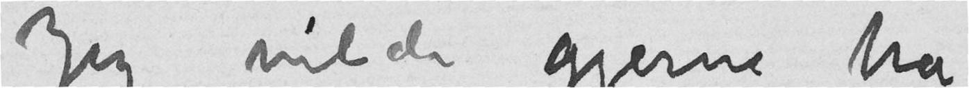Jeg vilde gjerne ha
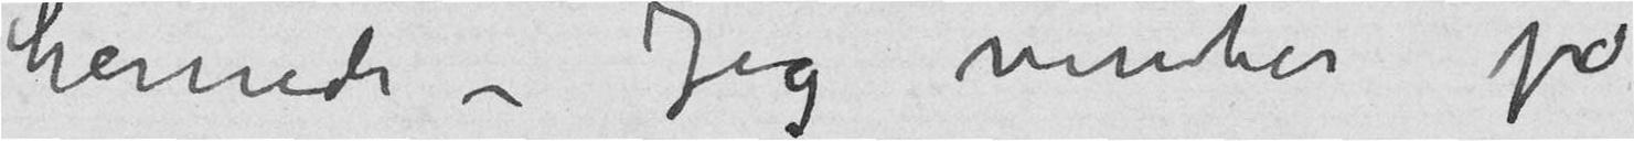hernede – Jeg venter jo
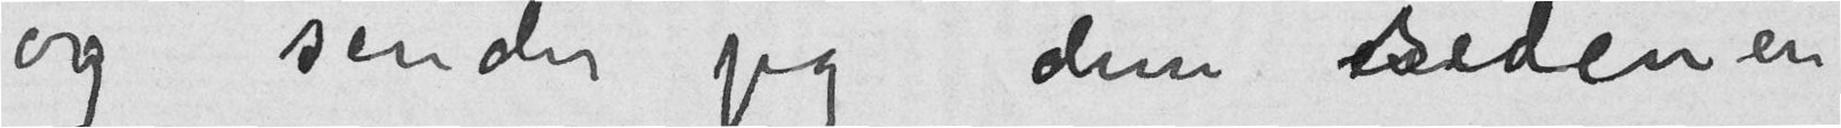og sender jeg dem siden en
